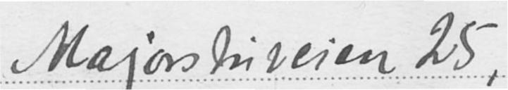Majorstuveien 25,
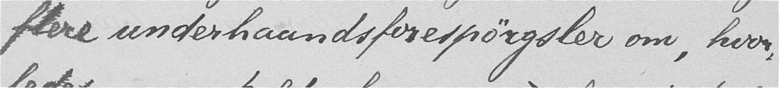flere underhaandsforespørgsler om, hvor-
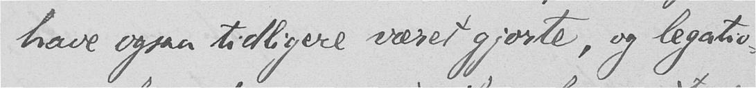have ogsaa tidligere været gjorte, og legatio-
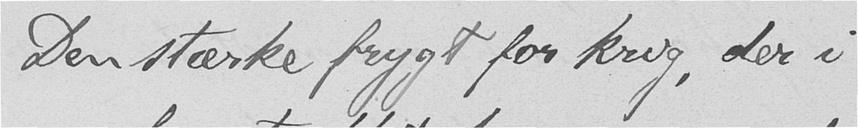Den stærke frygt for krig, der i
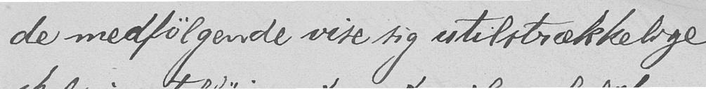de medfølgende vise sig ustilstrækkelige,
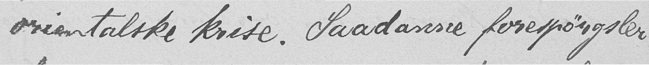orientalske krise. Saadanne forespørgsler
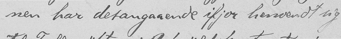nen har desangaaende ifjor henvendt sig
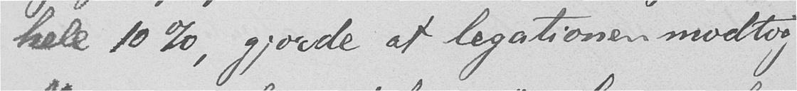hele 10%, gjorde at legationen modtog
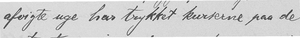afvigte uge har trykket kurserne paa de
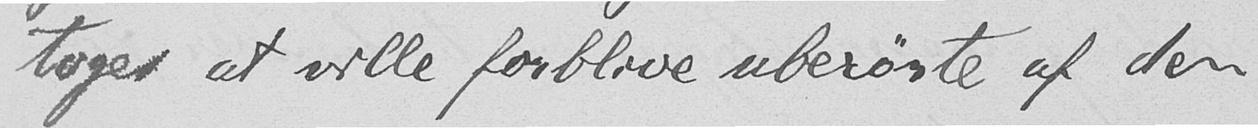tages at ville forblive uberørte af den
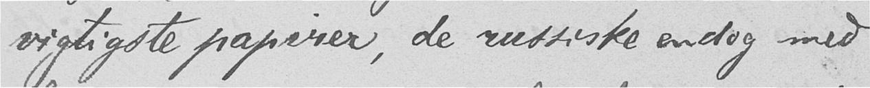vigtigste papirer, de russiske endog med
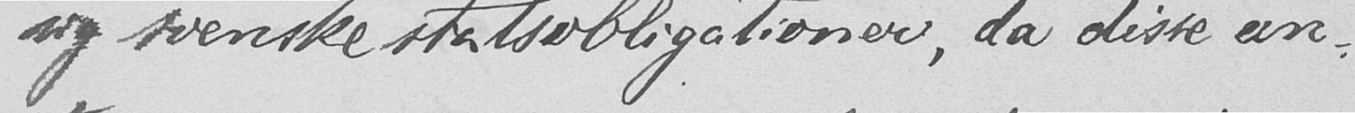sig svenske statsobligationer, da disse an-
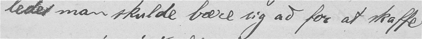ledes man skulde bære sig ad for at skaffe
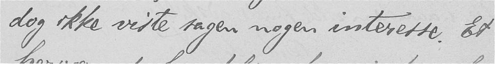dog ikke viste sagen nogen interesse. Et
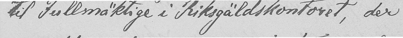til Fullmäktige i Riksgäldskontoret, der
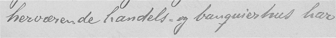herværende handels- og banquierhus har
